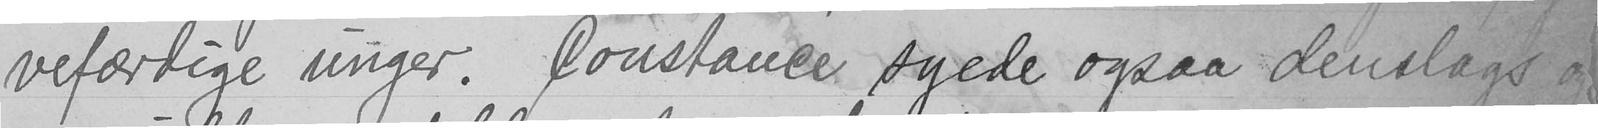vefærdige vinger. Constance syede ogsaa denslags og
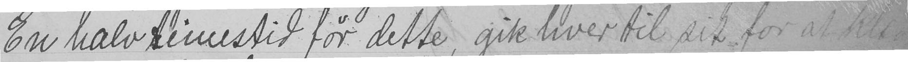En halv timestid før dette, gik hver til sit for at kle
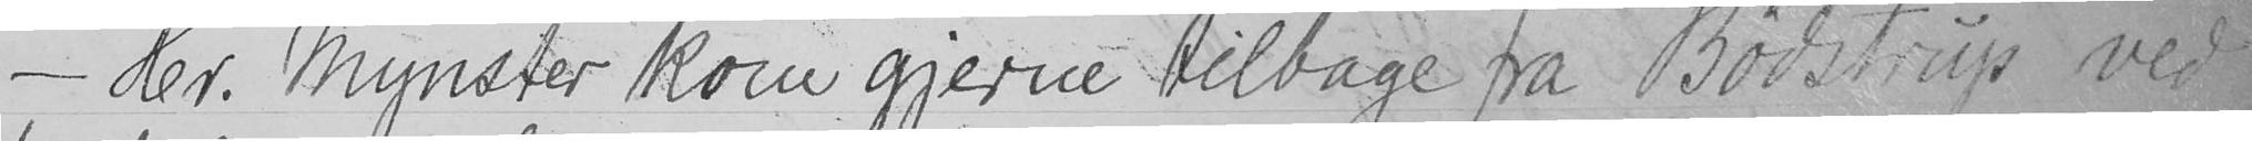- Her. Mynster kom gjerne tilbage fra Bødstrup ved
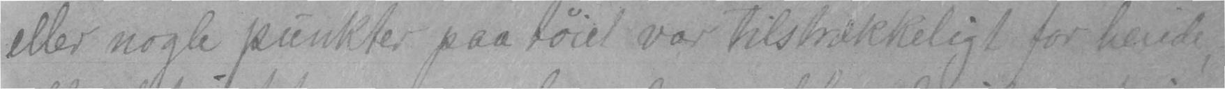eller nogle punkter paa tøiet var tilstrekkeligt for hende,
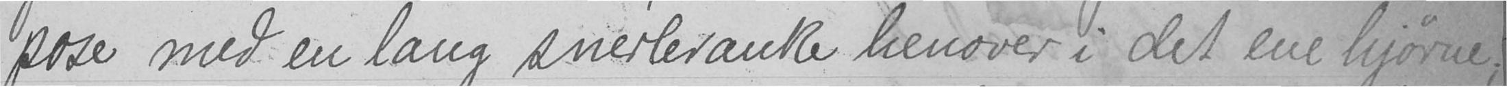pose med en lang snerleranke henover i det ene hjørne;
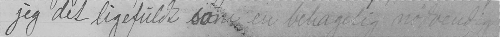jeg det ligefuldt som en behagelig nødvendighed
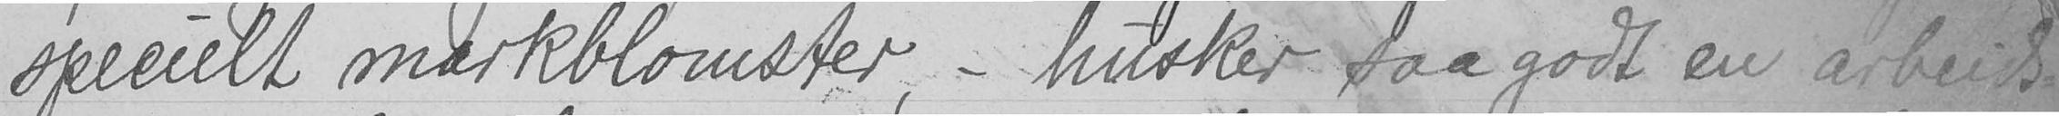specielt markblomster, - husker saa godt en arbeids-
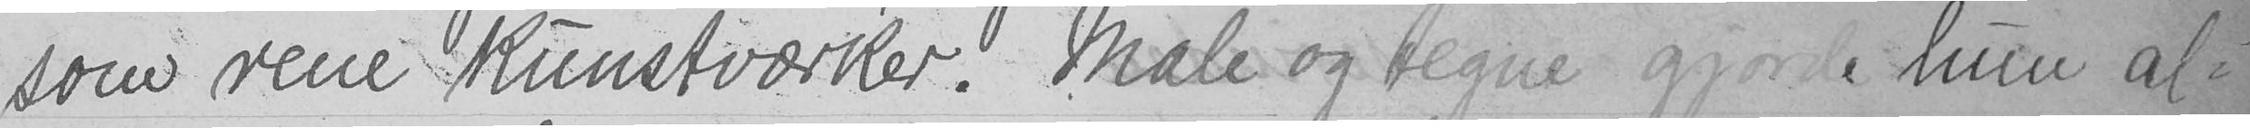som rene kunstværker. Male og tegne gjorde hun al-
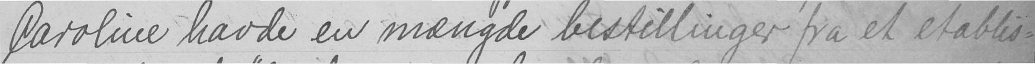Caroline havde en mængde bestillinger fra et etablis-
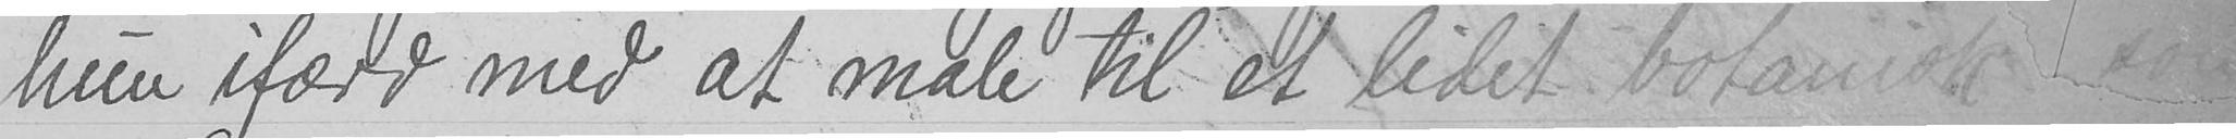hun ifærd med at male til et lidet botanisk
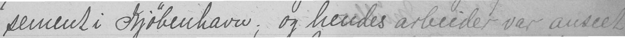sement i Kjøbenhavn; og hendes arbeider var anseet
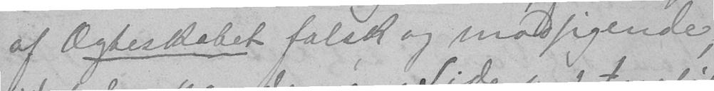af Ægteskabet falsk og modsigende,
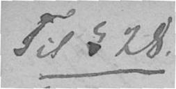Til § 28.
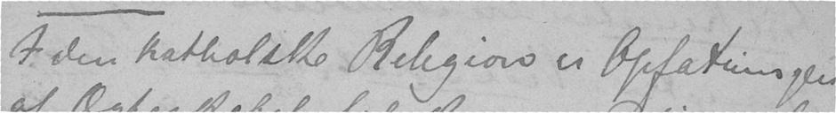I den katholske Religion er Opfatningen
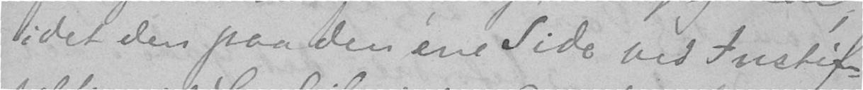idet den paa den ene Side ved Instif-
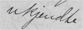ukjendte
